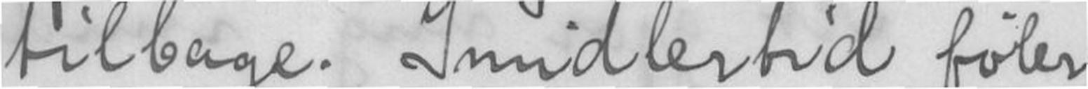tilbage. Imidlertid føler
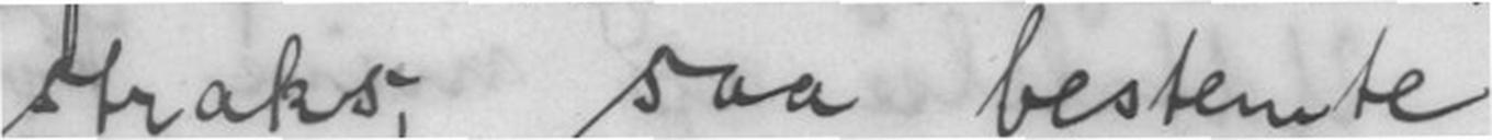straks, saa bestemte
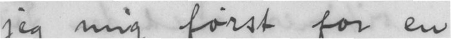jeg mig først for en
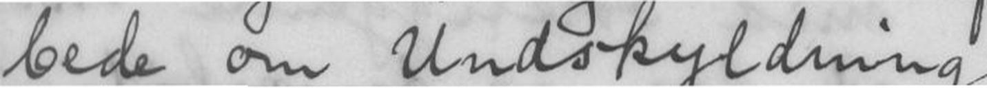bede om Undskyldning
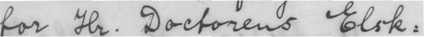for Hr. Doctorens Elsk-
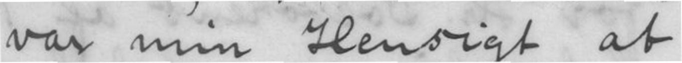var min Hensigt at
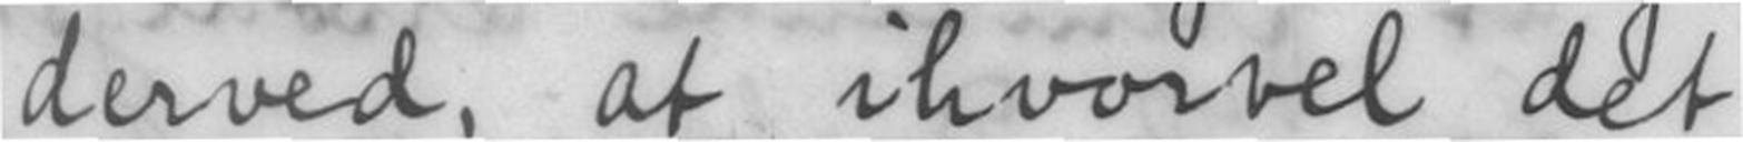derved, at ihvorvel det
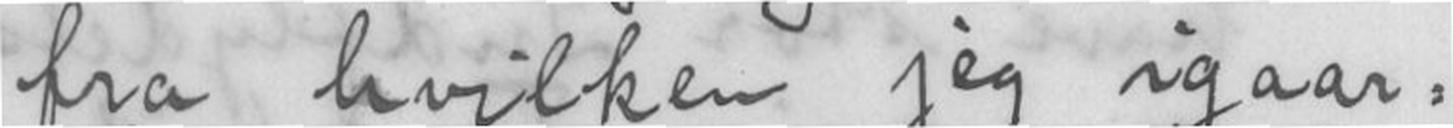fra hvilken jeg igaar-
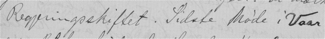Regjeringsskiftet. Sidste møde i vaar
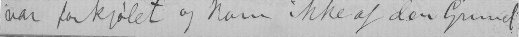var forkjølet og kom ikke af den Grund
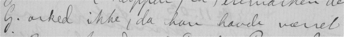G. orked ikke, da han havde værret
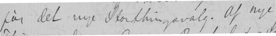før det nye Storthingsvalg. Af nye
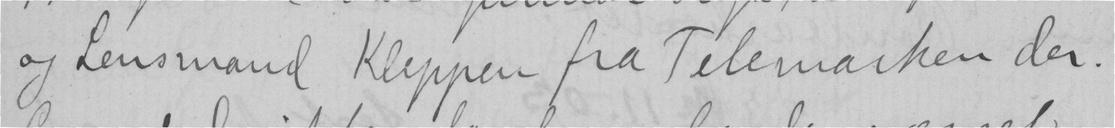og Lensmand Kleppen fra Telemarken der.
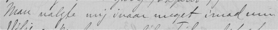Man valgte mig i vaar meget imod min
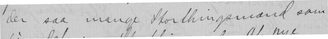der saa mange Storthingsmænd som
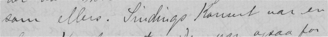som ellers. Sindings Konsert var en
